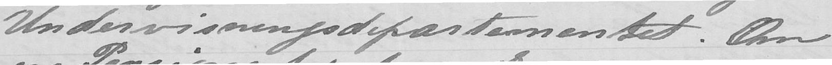Undervisningsdepartementet. Om
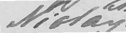Nicolay
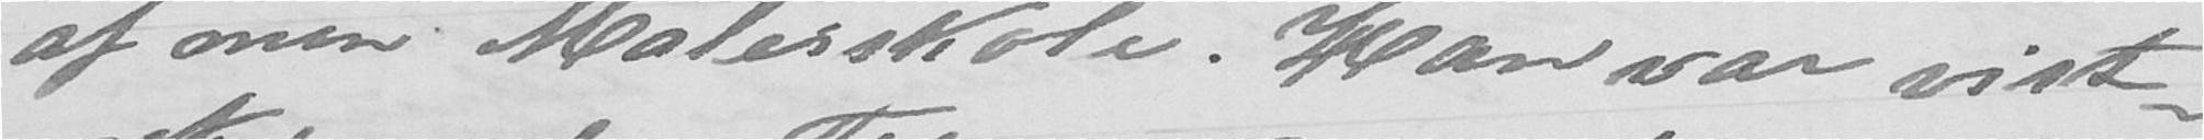af min Malerskole. Han var vist-
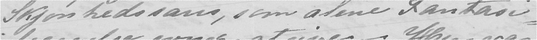Skjønhedssans, som alene Fantasi-
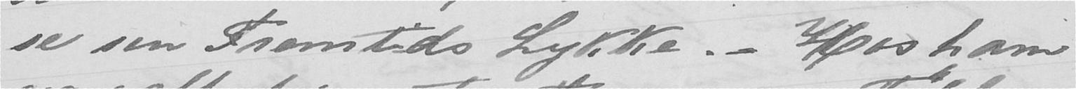se sin Fremtids Lykke. - Hos ham
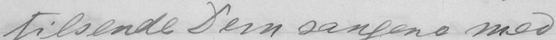tilsende Dem sangene med
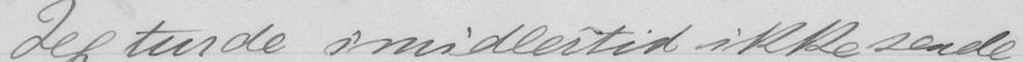Jeg turde imidlertid ikke sende
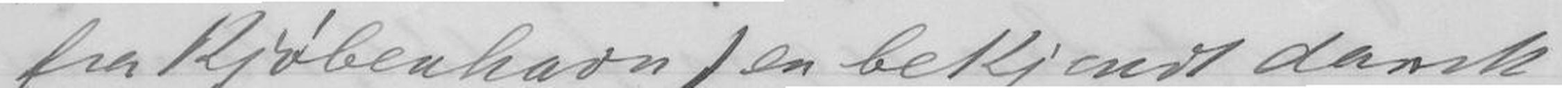fra Kjøbenhavn) en bekjendt dansk
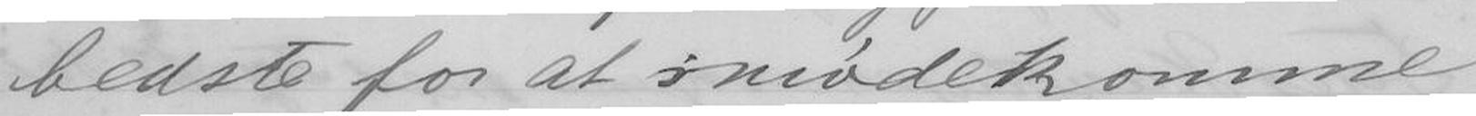bedste for at imødekomme
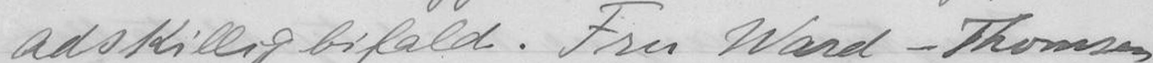adskillig bifald. Fru Ward-Thomsen
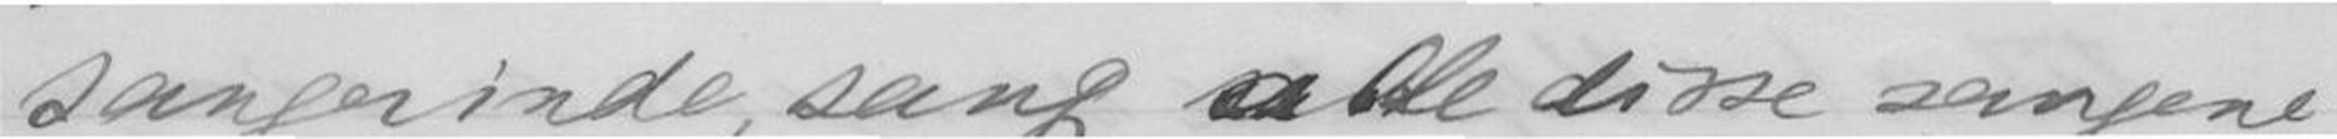sangerinde, sang alle disse sangene
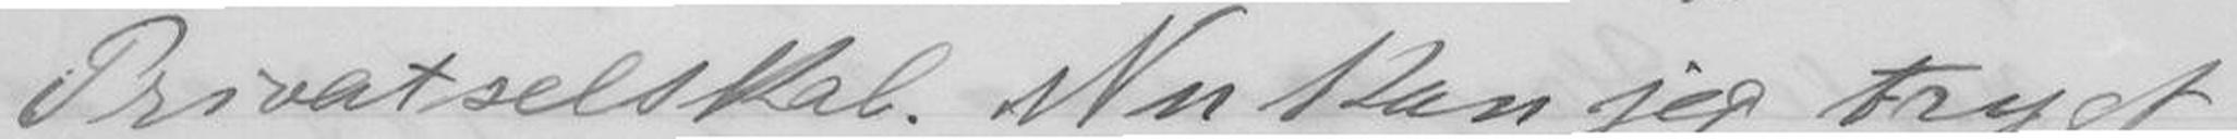Privatselskab. Nu kan jeg trygt
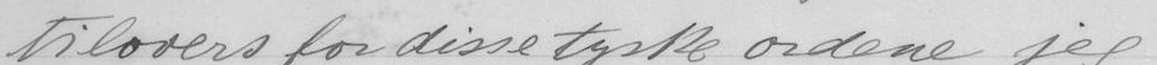tilovers for disse tyske ordene jeg
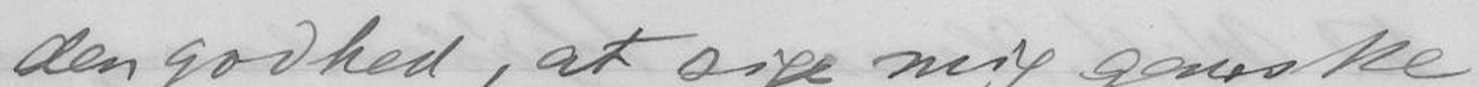den godhed, at sige mig ganske
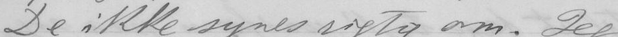De ikke synes rigtig om. Jeg
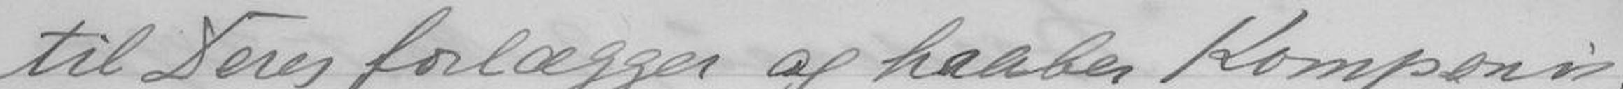til Deres forlægger af og haaber Komponis-
